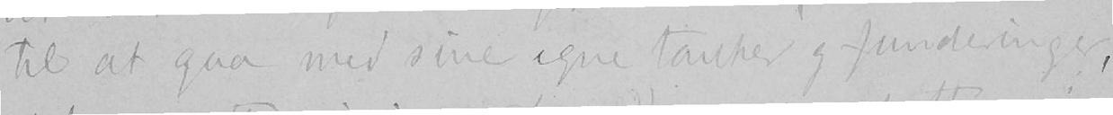til at gaa med sine egne tanker og funderinger,
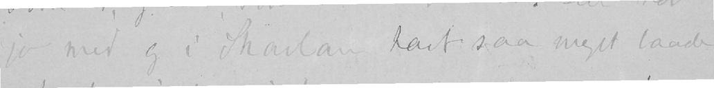jo med og i Skavlan havt saa meget baade
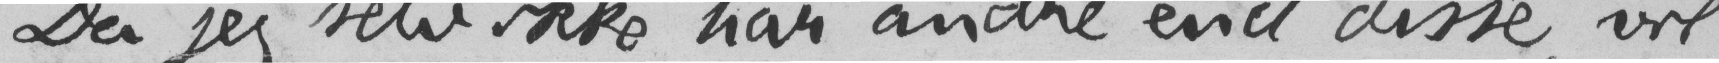Da jeg selv ikke har andre end disse, vil
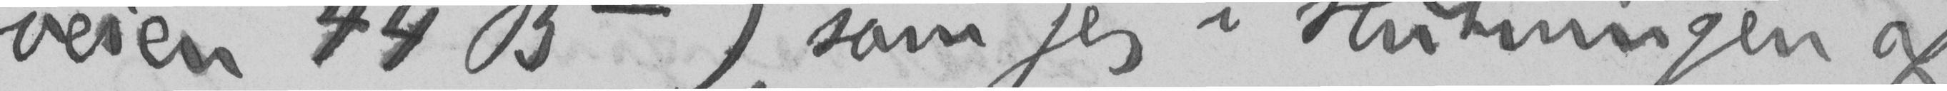veien 44 B IV), som jeg i slutningen af
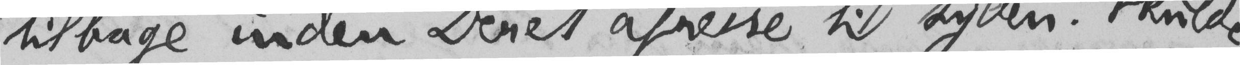tilbage inden Deres afreise til syden. Skulde
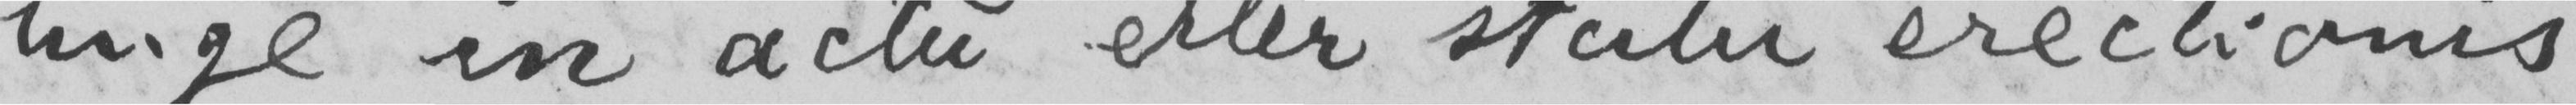linge in actu eller statu erectionis,
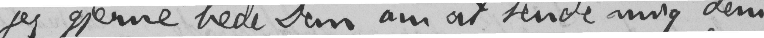jeg gjerne bede Dem om at sende mig dem
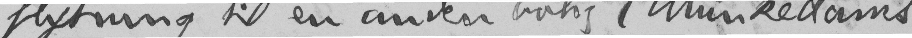flytning til en anden bolig (Munkedams-
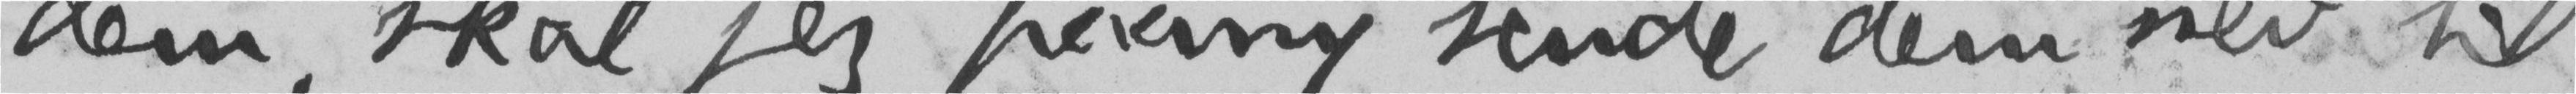dem, skal jeg paany sende dem ned til
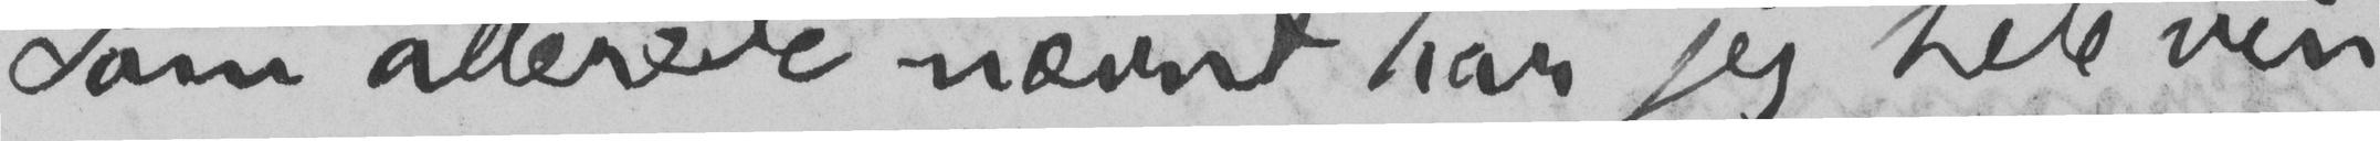Som allerede nævnt har jeg hele vin-
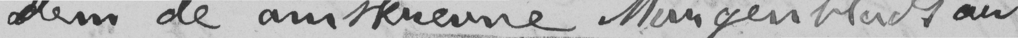Dem de omskrevne Morgenbladsar-
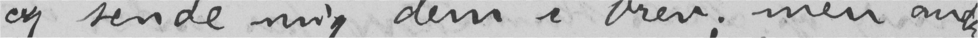og sende mig dem i brev; men andre
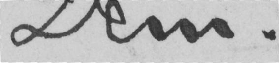Dem.
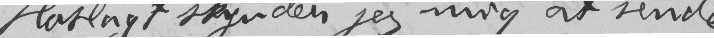Hoslagt skynder jeg mig at sende
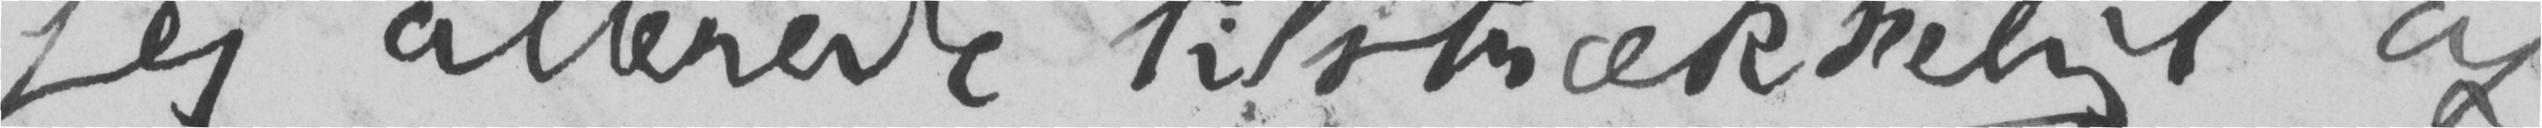jeg allerede tilstrækkeligt af.
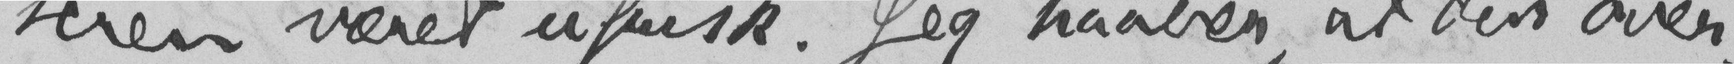teren været ufrisk. Jeg haaber, at den over-
